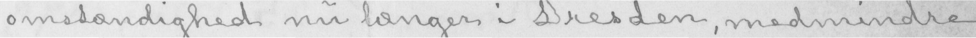omstændighed nu længer i Dresden, medmindre
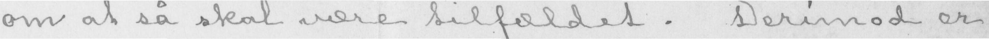om at så skal være tilfældet. Derimod er
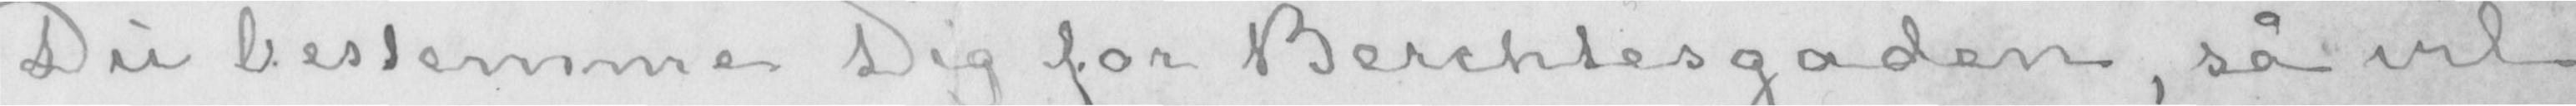Du bestemme Dig for Berchtesgaden, så vil
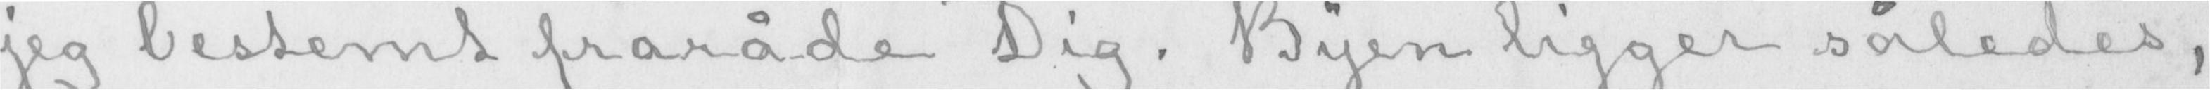jeg bestemt fraråde Dig. Byen ligger således,
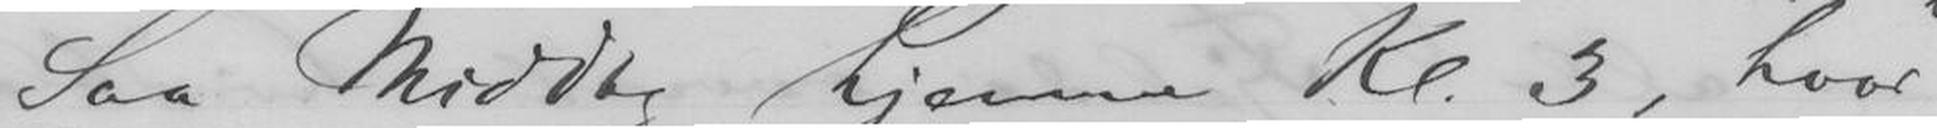Saa Middag hjemme Kl. 3, hvor
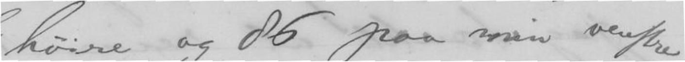høire og 86 paa min venstre
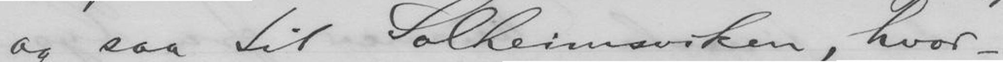og saa til Solheimsviken, hvor-
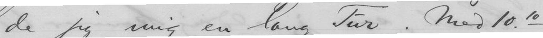de jeg mig en lang Tur. Med 10.10
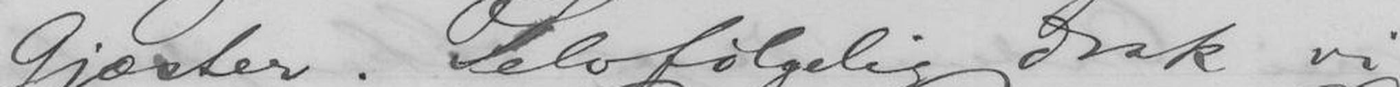Gjæster. Selvfølgelig drak vi
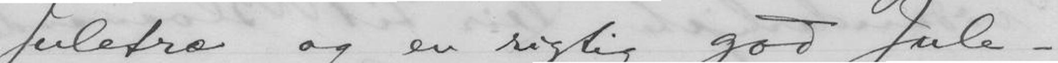Juletræ og en rigtig god Jule-
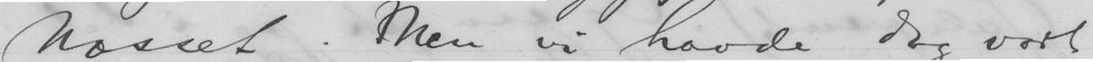Næsset. Men vi havde dog vort
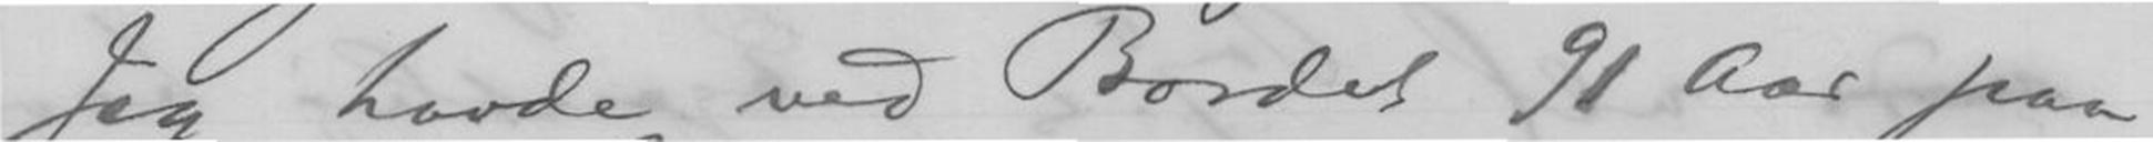Jeg havde ved Bordet 91 Aar paa
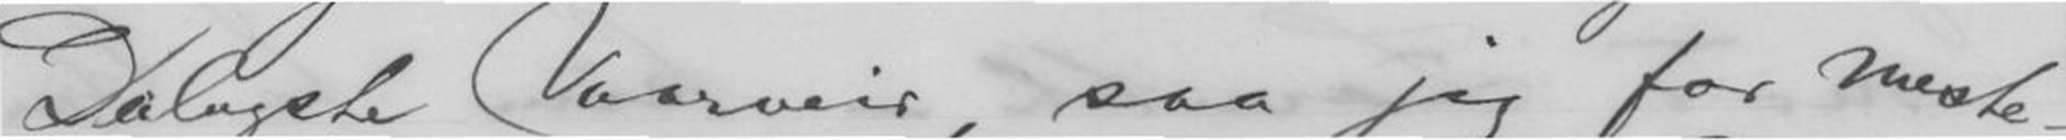Deiligste Vaarveir, saa jeg for Meste-
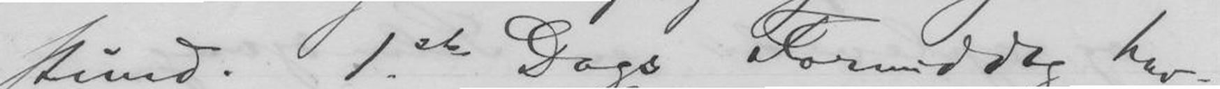stund. 1ste Dags Formiddag hav-
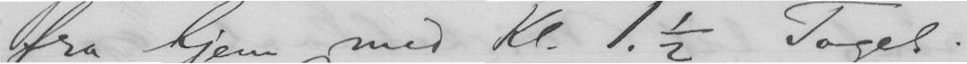fra hjem med Kl. 1.½ Toget.
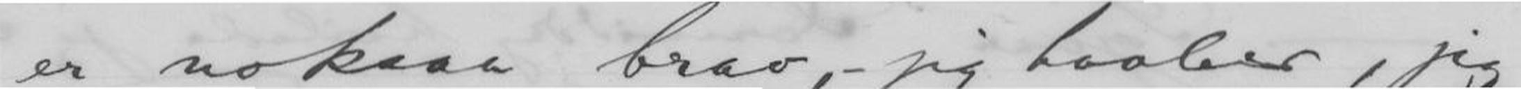er noksaa brav, - jeg haaber, jeg
